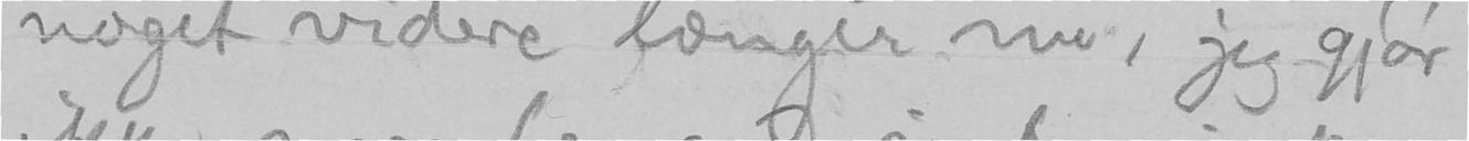noget videre længer nu, jeg gjør
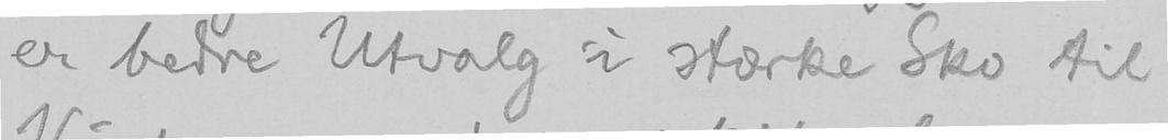er bedre Utvalg i stærke Sko til
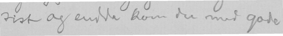sist og endda kom du med gode
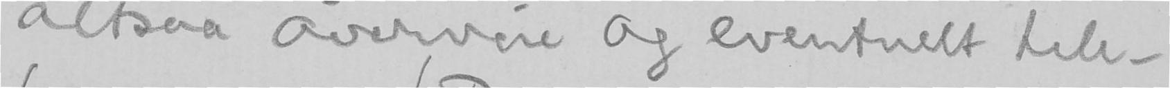altsaa overveie og eventuelt tele-
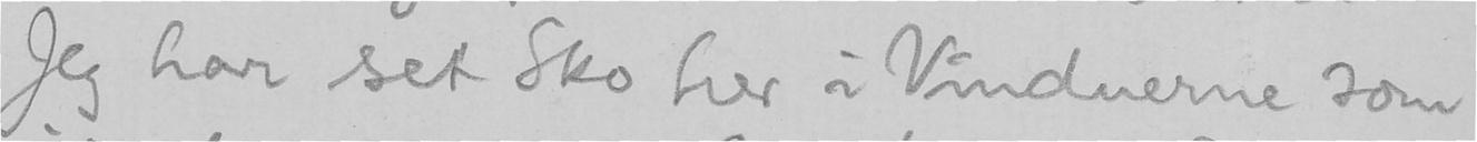Jeg har set Sko her i Vinduerne som
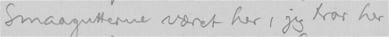Smaagutterne været her, jeg tror her
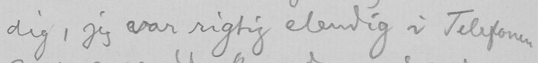dig, jeg var rigtig elendig i Telefonen
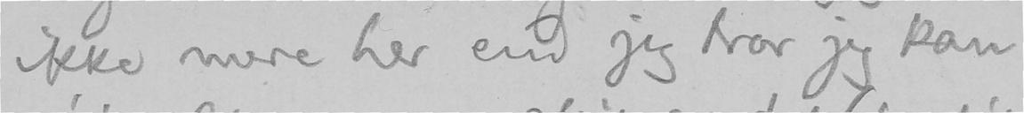ikke mere her end jeg tror jeg kan
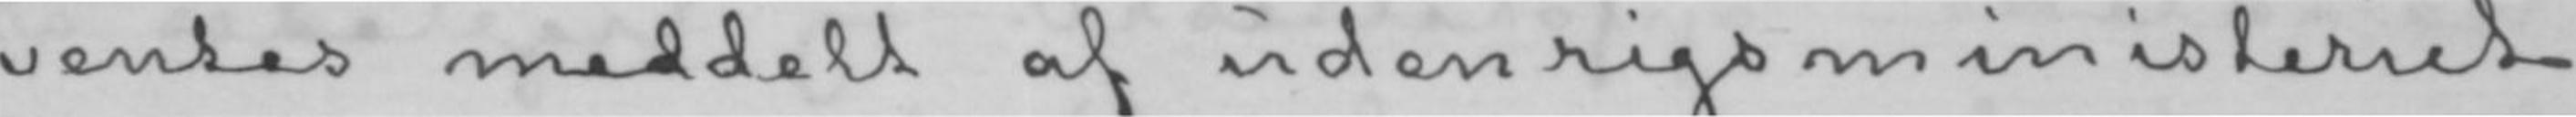ventes meddelt af udenrigsministeriet,
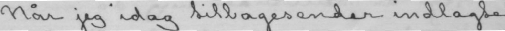Når jeg idag tilbagesender indlagte
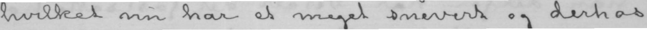hvilket nu har et meget snevert og derhos
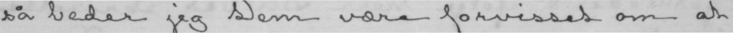så beder jeg Dem være forvisset om at
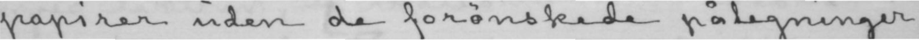papirer uden de forønskede påtegninger,
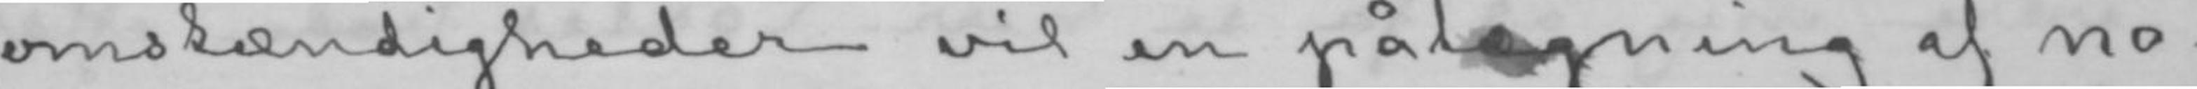omstændigheder vil en påtegning af no-
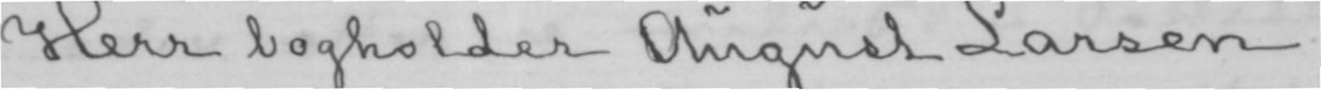Herr bogholder August Larsen.
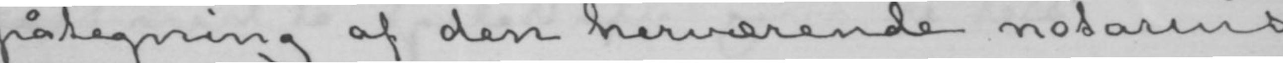påtegning af den herværende notarius
